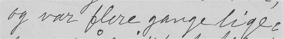og var flere gange lige-
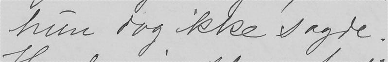hun dog ikke sagde.
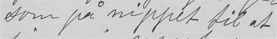som på nippet til at
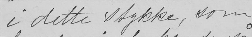i dette stykke, som
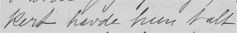kert havde hun talt
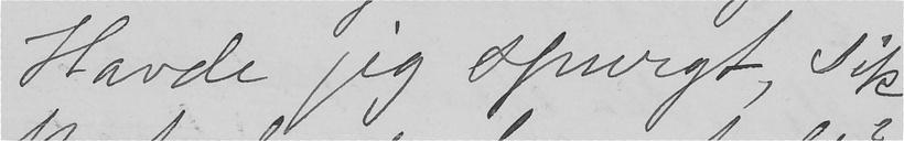Havde jeg spurgt, sik-
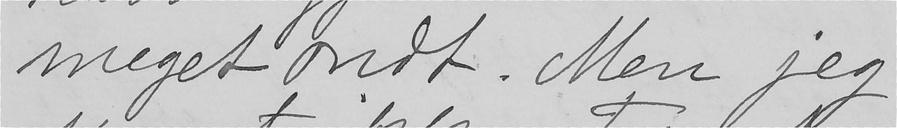meget ondt. Men jeg
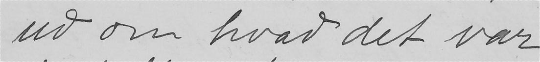ud om hvad det var
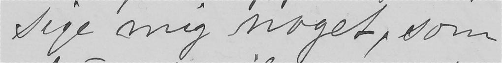sige mig noget, som
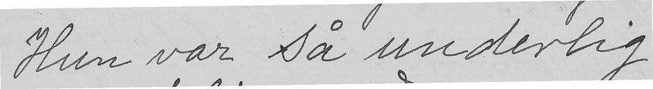Hun var så underlig
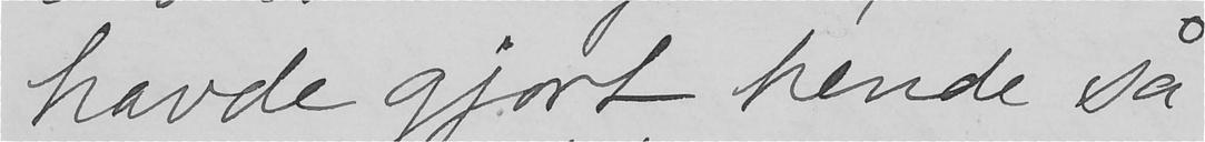havde gjort hende så
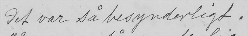Det var så besynderligt.
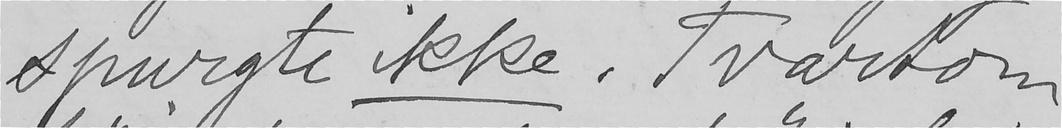spurgte ikke. Tværtom
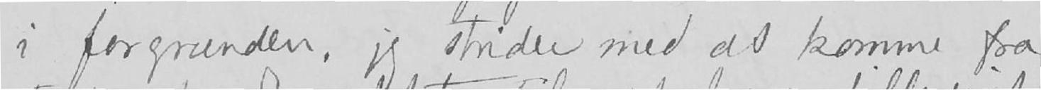i forgrunden, jeg strider med at komme fra
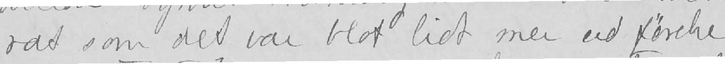rat som det var blot lidt mer udførelse
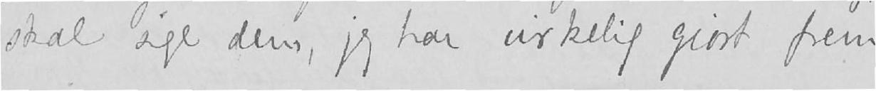skal sige dem, jeg har virkelig giort frem-
